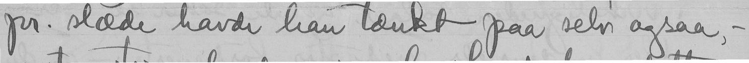pr. slæde havde han tænkt paa selv ogsaa, -
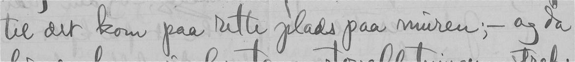til det kom paa rette plads paa muren; - og da
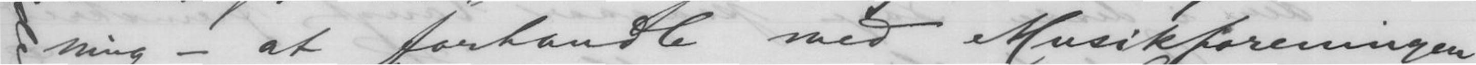ning - at forhandle med Musikforeningen
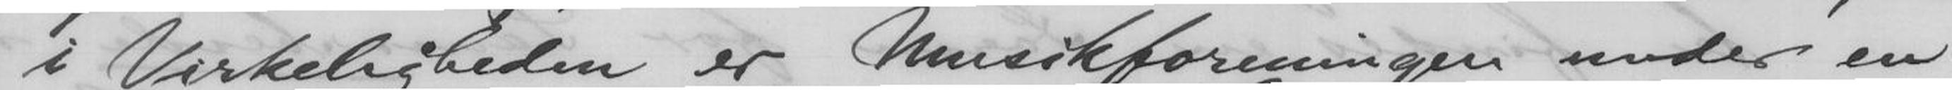i Virkeligheden er Musikforeningen under en
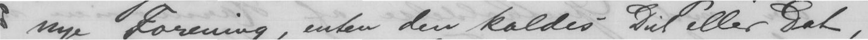nye Forening, enten den kaldes Dit eller Dat,
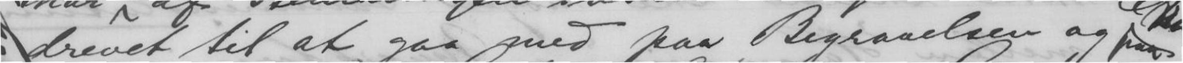drevet til at gaa med paa Begravelsen og paa
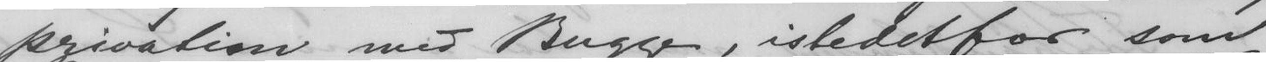privatim med Bugge, istedetfor som
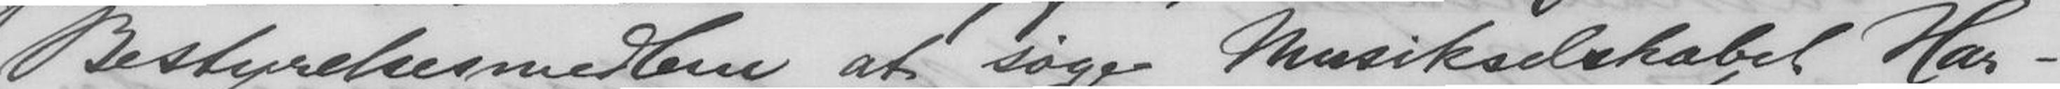Bestyrelsemedlem at søge Musikselskabet Har-
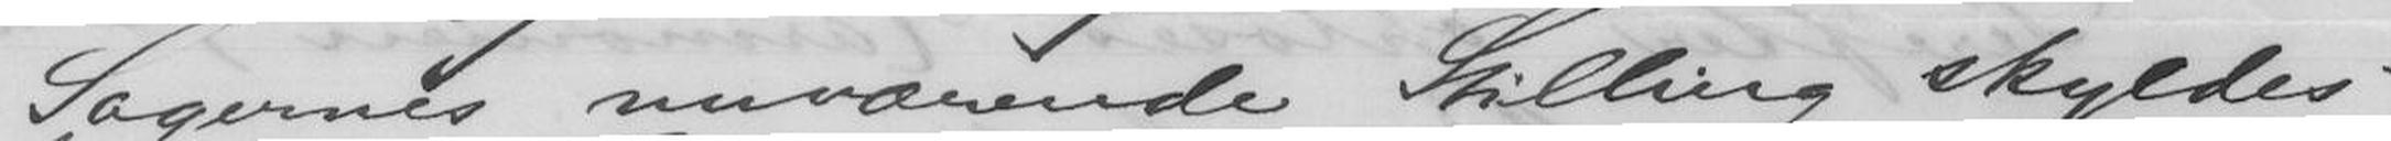Sagernes nuværende Stilling skyldes
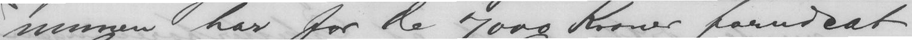munen har for de 7000 Kroner forudsat
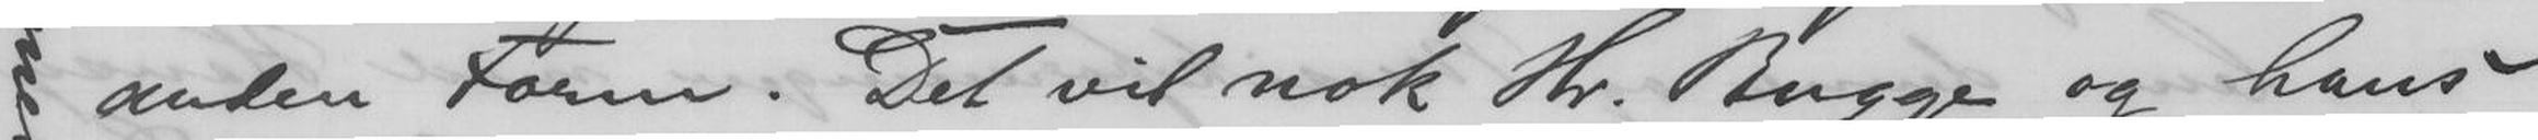anden Form. Det vil nok Hr. Bugge og hans
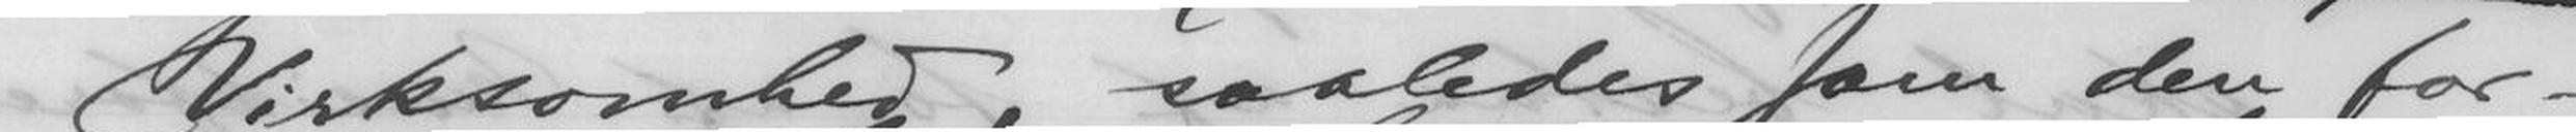Virksomhed, saaledes som den for-
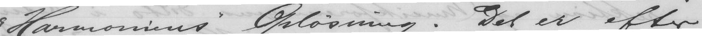"Harmoniens" Opløsning. Det er efter
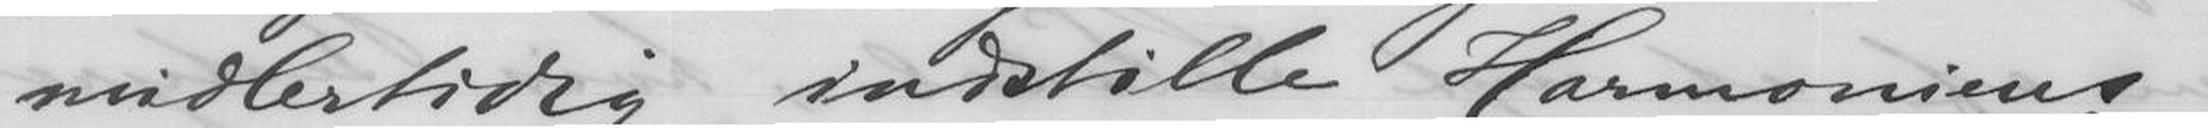midlertidig indstille Harmoniens
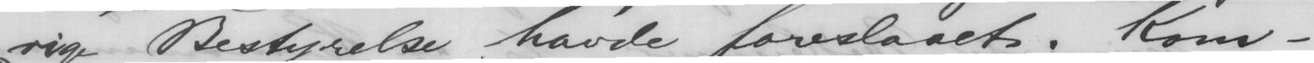rige Bestyrelse havde foreslaaet. Kom-
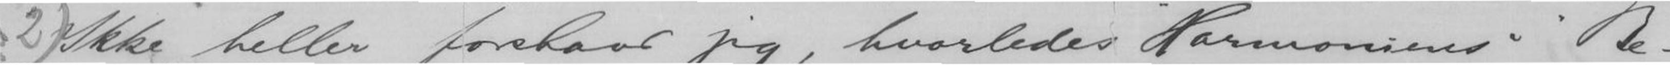Ikke heller forstaar jeg, hvorledes "Harmoniens" Be-
